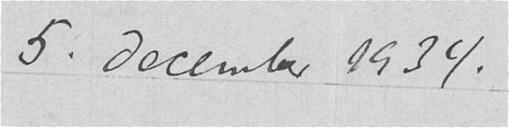5. december 1934.
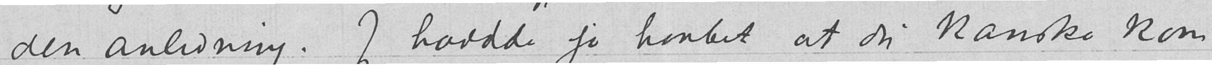den anledning. Jeg hadde jo haabet at du kanske kom
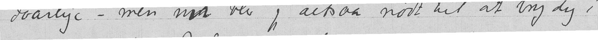daarlige – men nu blir jeg altsaa nødt til at bry dig i
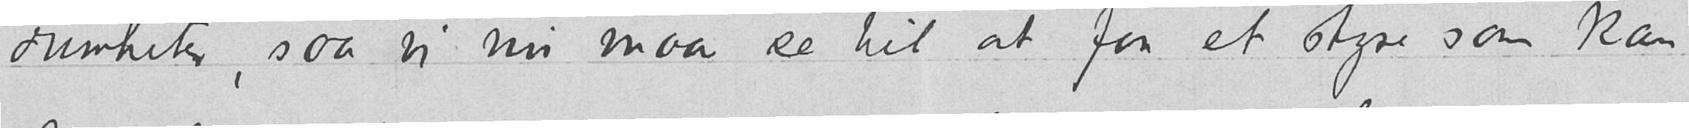dumheter, saa vi nu maa se til at faa et styre som kan
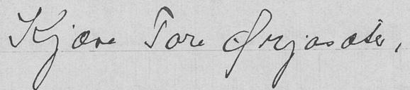Kjære Tore Ørjasæter,
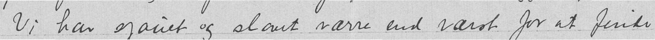Vi har sjauet og slavet værre end værst for at finde
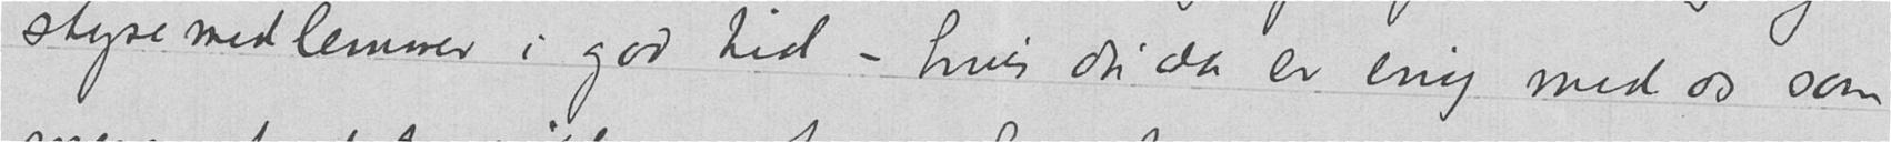styremedlemmer i god tid – hvis du da er enig med os som
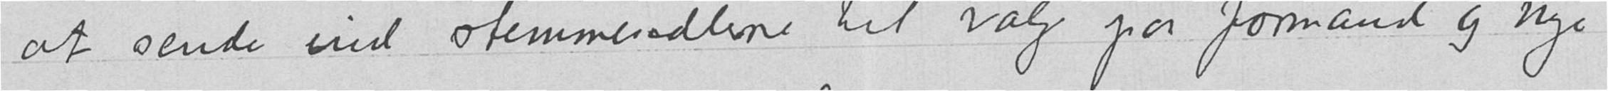at sende ind stemmesedlene til valg paa formand og nye
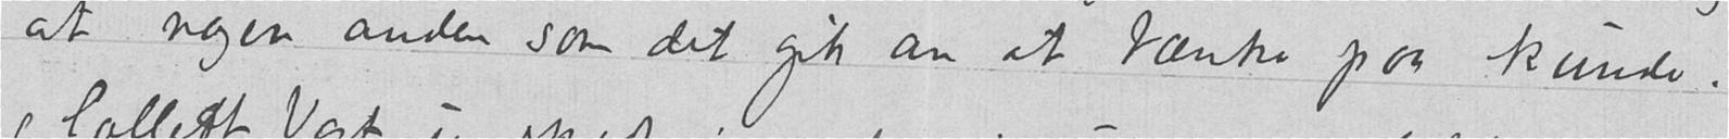at nogen anden som det gik an at tænke paa kunde.
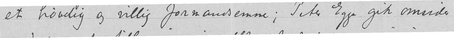et høvelig og villig formandsemme; Peter Egge gik omsider
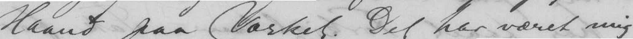Haand paa Værket. Det har været mig
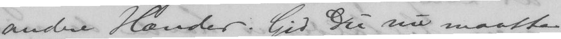andre Hænder. Gid Du maatte
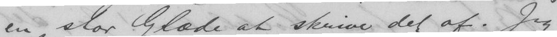en stor Glæde at skrive det af. Jeg
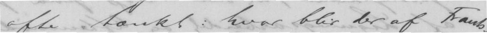ofte tænkt: hvor blir der af Frants?
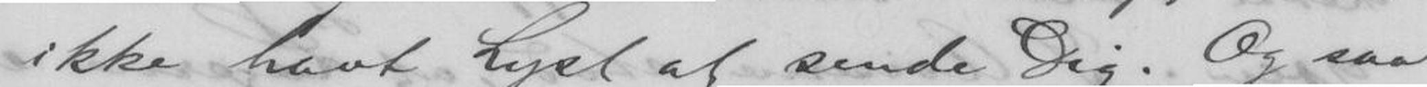ikke havt Lyst at sende Dig. Og saa
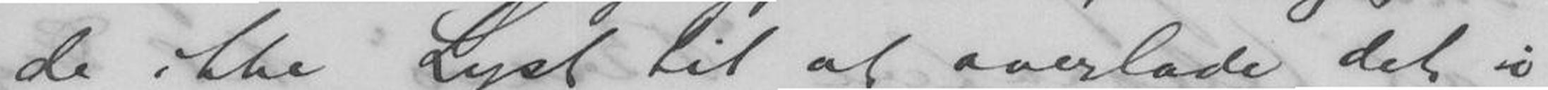de ikke Lyst til at overlade det i
